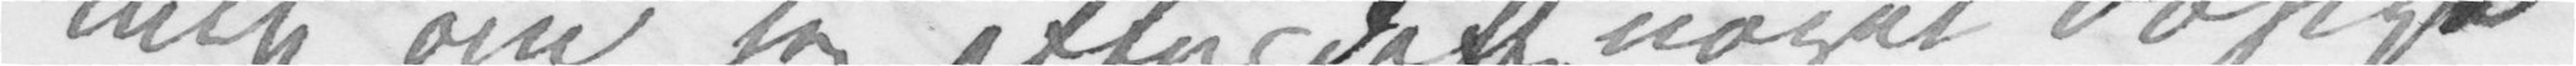mig om jeg efter dette noget døsige
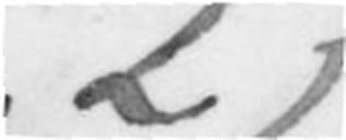2
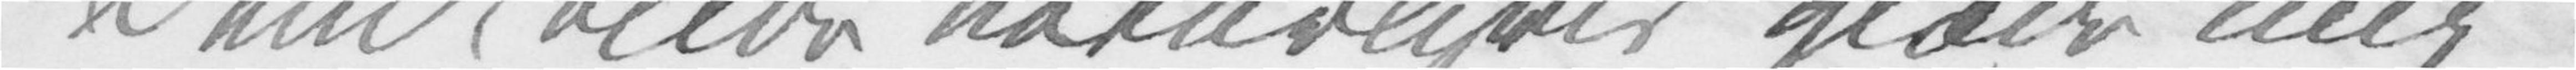Dem vilde naturligvis glæde mig
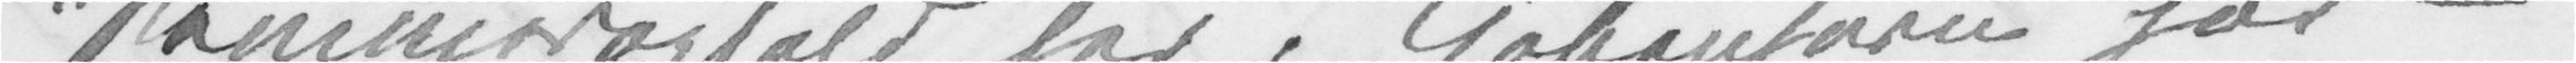Sommerophold her i Kjøbenhavn har
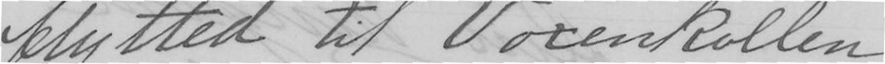flytted til Voxenkollen
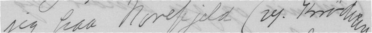jeg paa Norefjeld (v/. Krøderen).
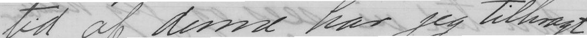tid af denne har jeg tilbragt
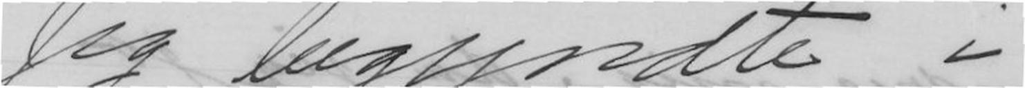Jeg begyndte i
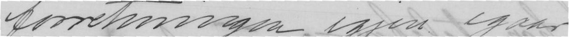forretningen igjen igaar
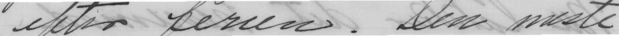efter ferien. Den meste
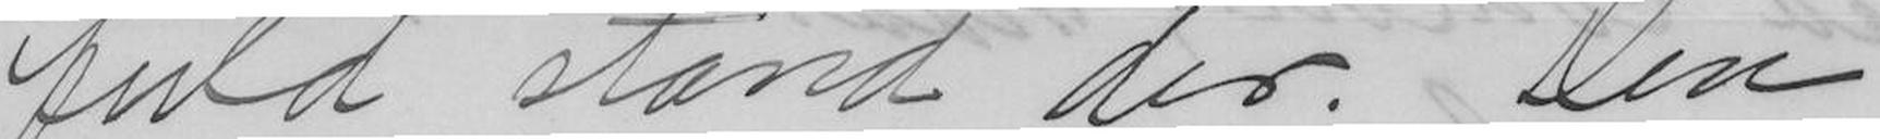fuld stand der. Den
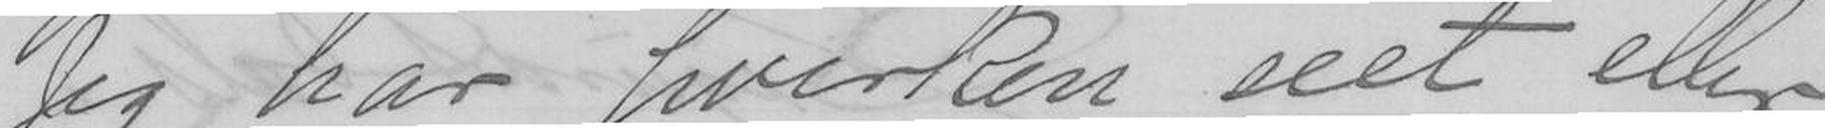Jeg har hverken seet eller
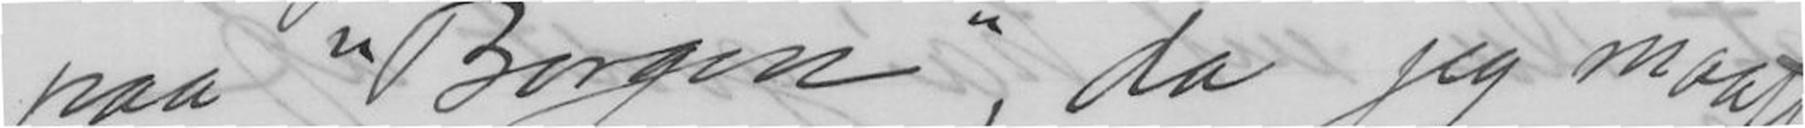paa "Borgen", da jeg maatte
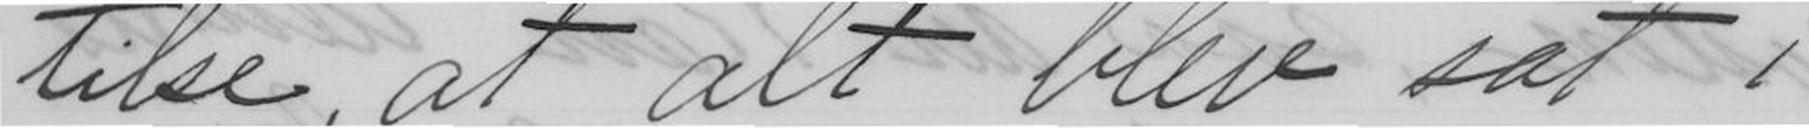tilse, at alt blev sat i
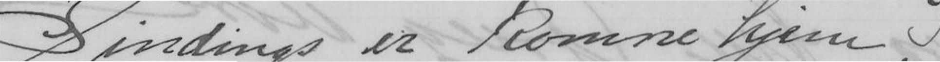Sindings er komne hjem,
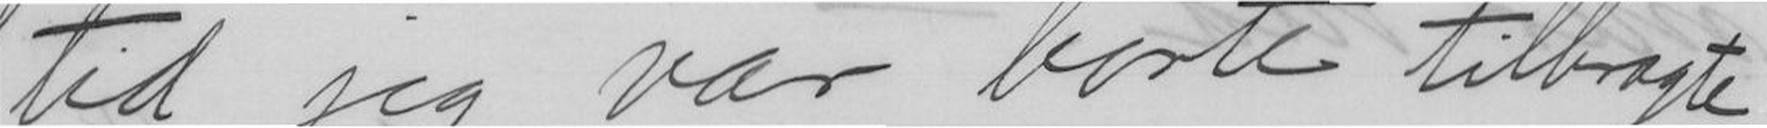tid jeg var borte tilbragte
